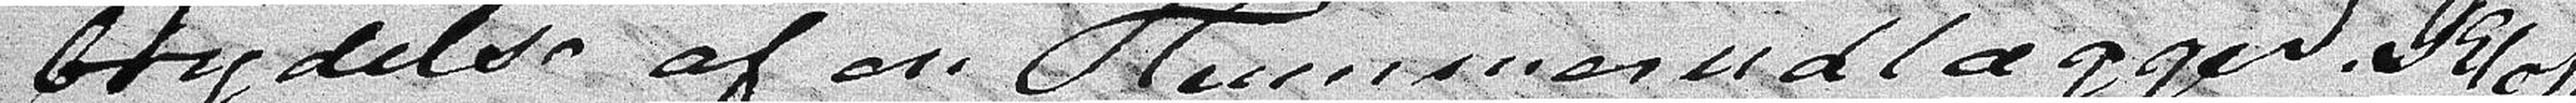brytelse af en Hummer udlægger. Klok-
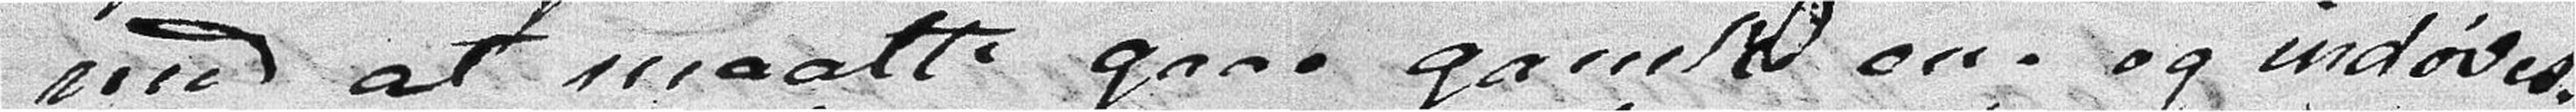med at maatte gaae ganske ene og indøves.
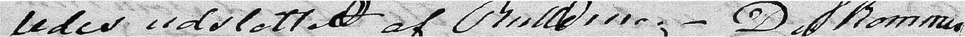ledes udslettet af Rullerne. - Du kommer
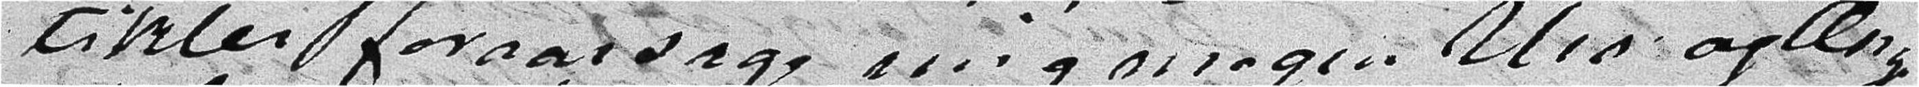tikler foraarsage mig megen Uro og Æng-
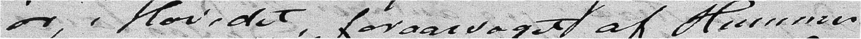ør i Hovedet, foraarsaget af Hummer
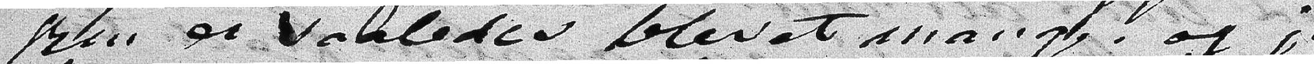ken er saaledes blevet mange, og jeg
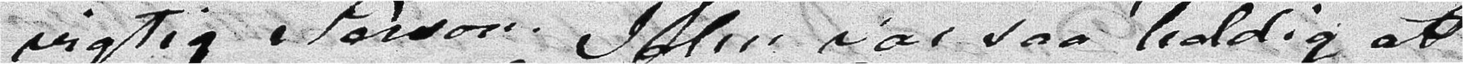vigtig Person. John var saa heldig at
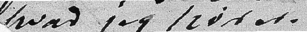hvad jeg hører.
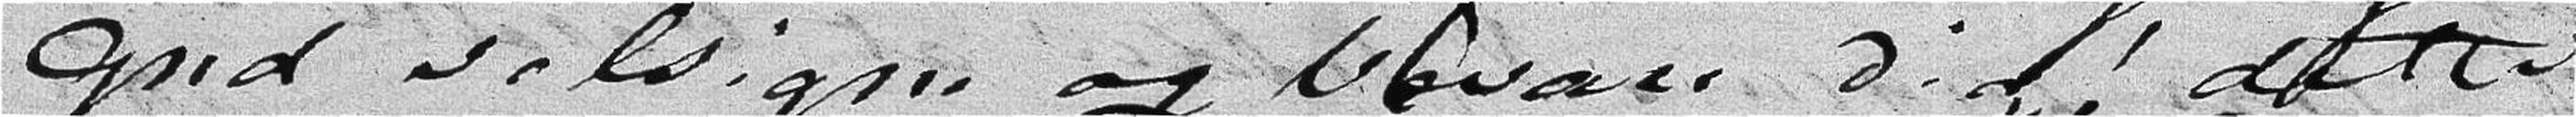Gud velsigne og bevare Dig! dette
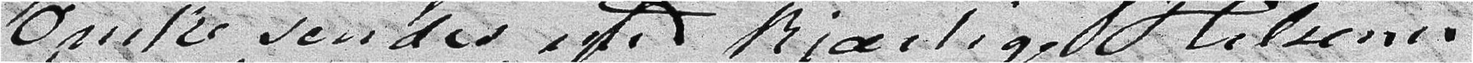Ønske sendes med kjærlige Hilsener
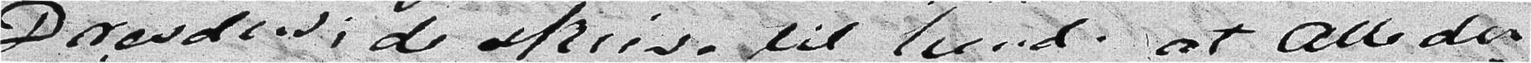Dresden; de skrive til hende at Alle der
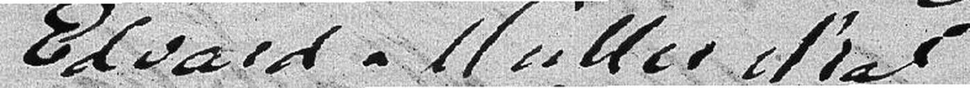Edvard Müller skal
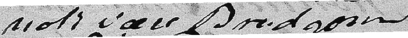nok være Brudgom
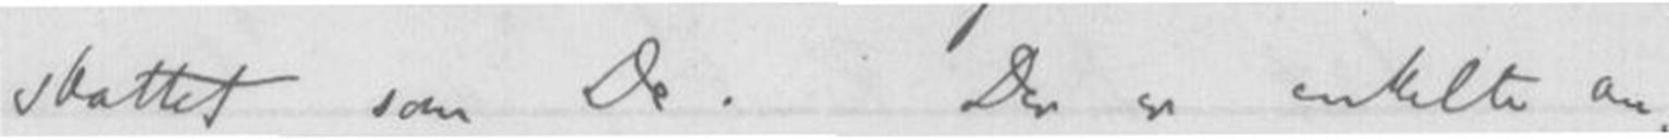og skattet som De. Der er enkelte an-
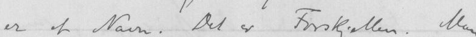er et Navn. Det er Forskjellen. Man
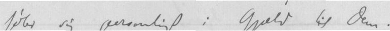føler sig personligt i gjæld til Dem.
